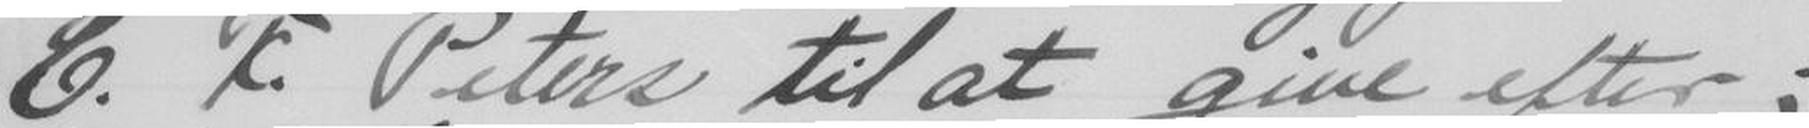C. F. Peters til at give efter;
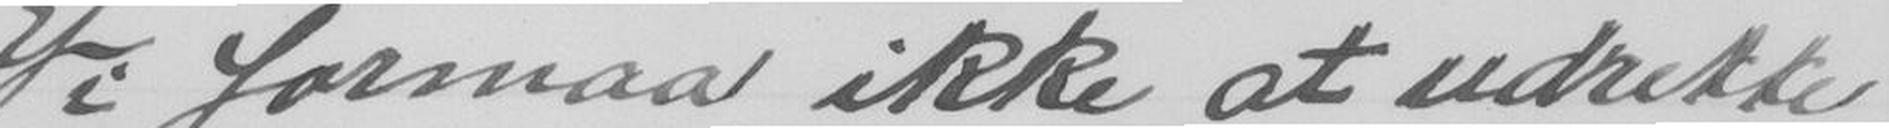Vi formaa ikke at udrette
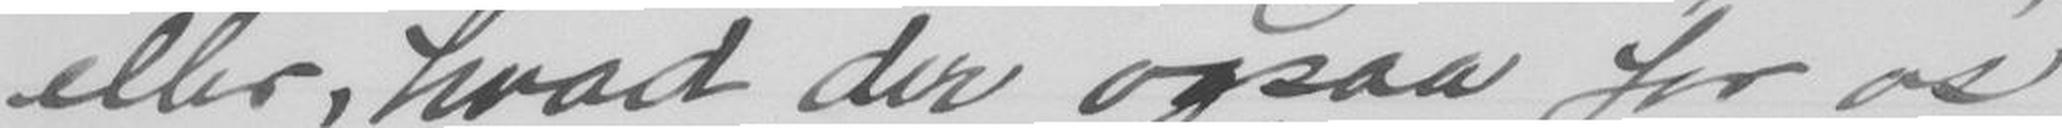eller, hvad der ogsaa for os
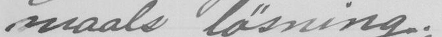maals løsning.
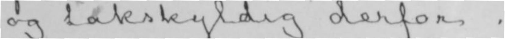og takskyldig derfor.
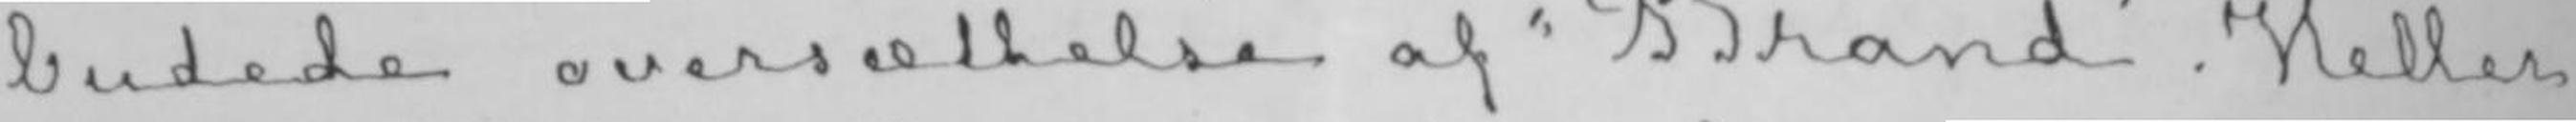budede oversættelse af «Brand». Heller
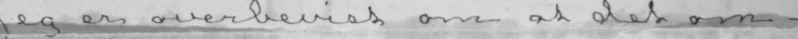Jeg er overbevist om at det om-
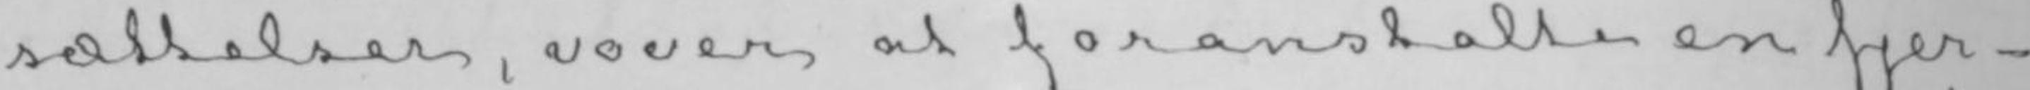sættelser, vover at foranstalte en fjer-
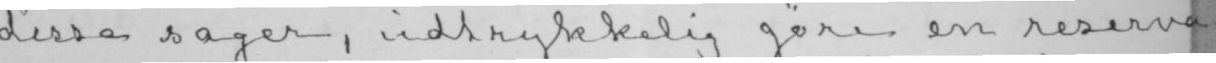disse sager, udtrykkelig gøre en reserva-
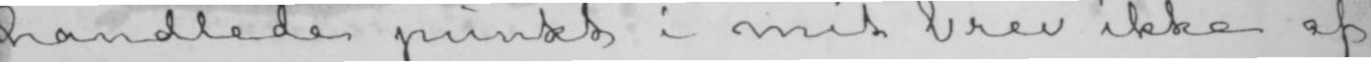handlede punkt i mit brev ikke af
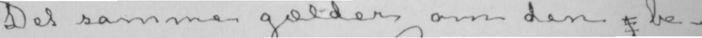Det samme gælder om den be-
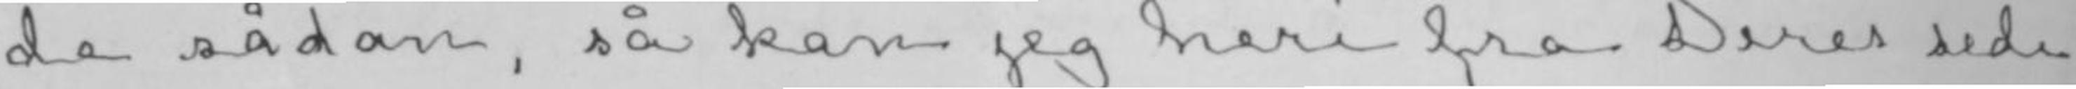de sådan, så kan jeg heri fra Deres side
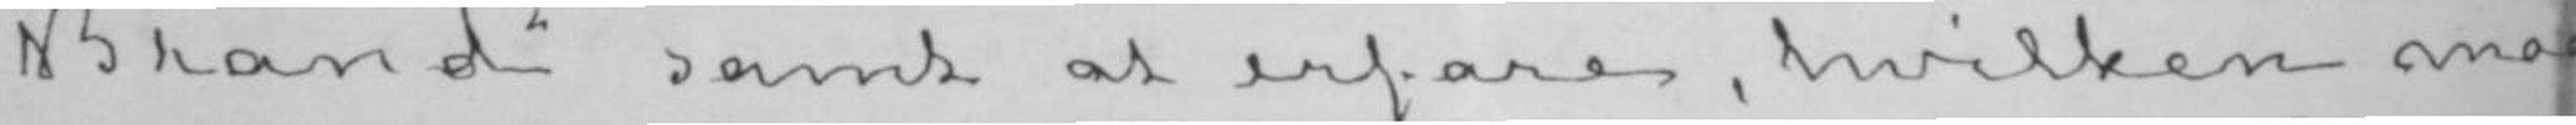«Brand» samt at erfare, hvilken mod-
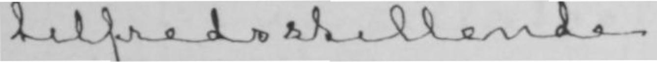tilfredsstillende.
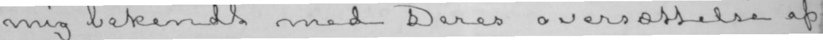mig bekendt med Deres oversættelse af
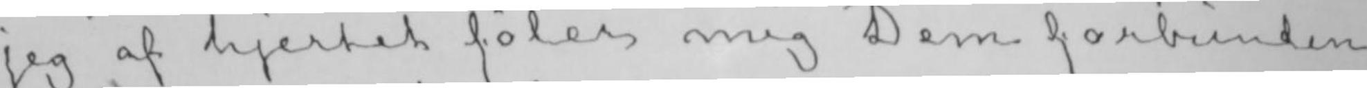jeg af hjertet føler mig Dem forbunden
